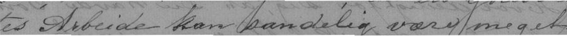tes Arbeide kan sandelig være meget
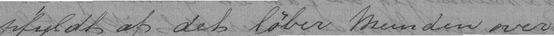opfyldt af, det løber Munden over med;
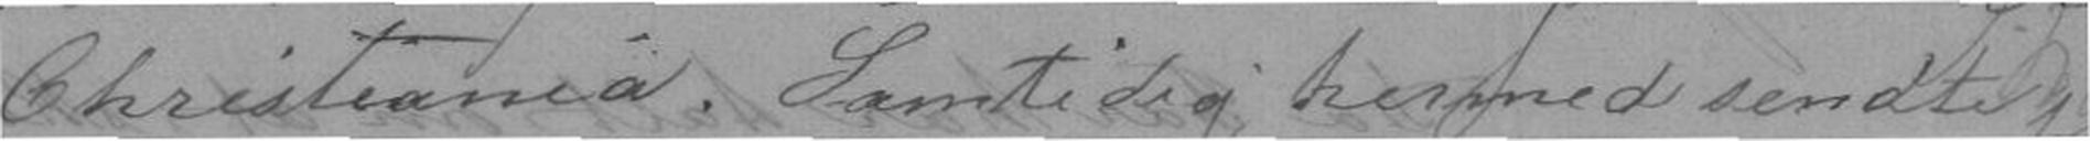Christiania. Samtidig hermed sendte jeg
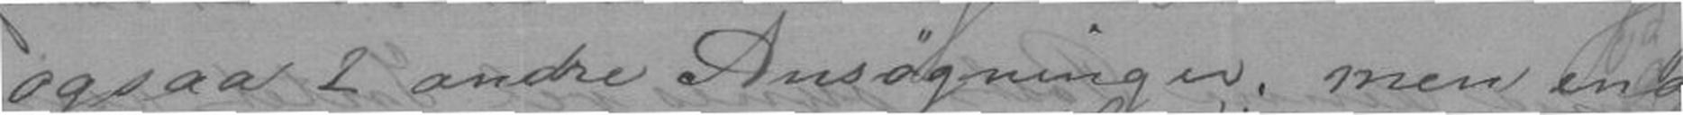ogsaa 2 andre Ansøgninger; men end-
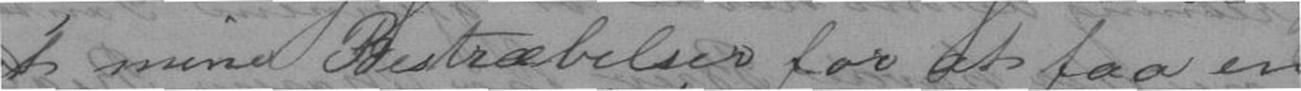at mine Bestræbelser for at faa en
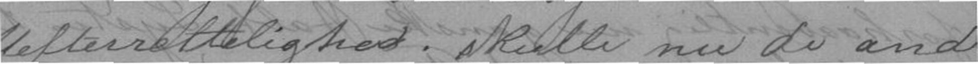Uefterettelighed; skulle nu de andre
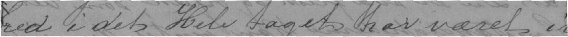bred i det Hele taget har været ivin-
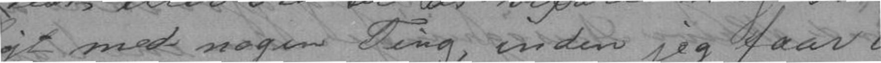ligt med nogen Ting, inden jeg faar
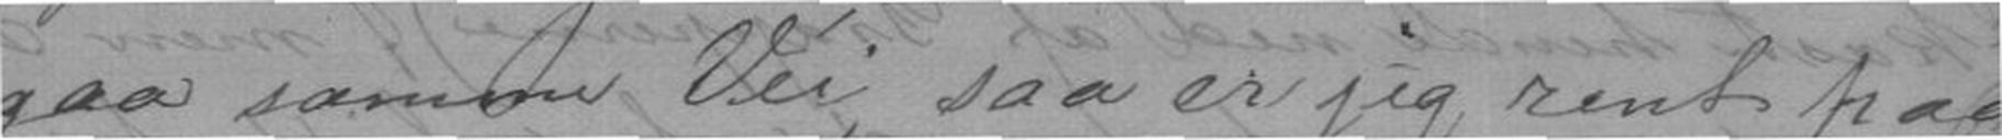gaa samme Vei, saa er jeg rent paa
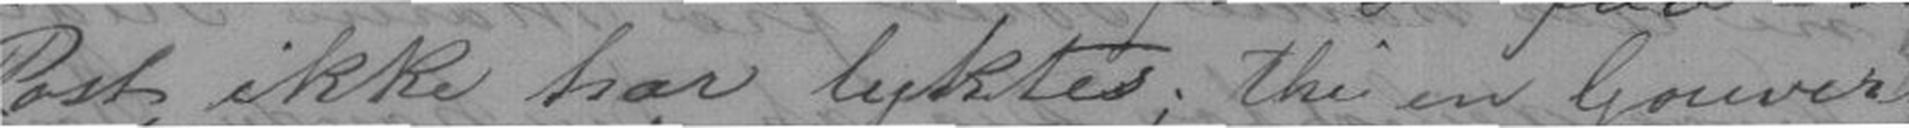Post ikke har lyktes; thi en Gouver
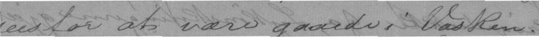sees for at være gaaede i Vasken.
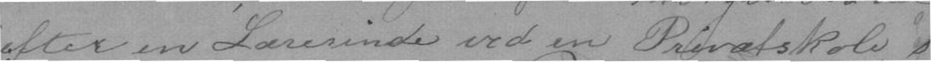efter en Lærerinde ved en Privatskole, som
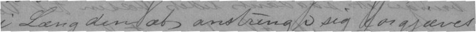i Længden at anstrenge sig forgjæves
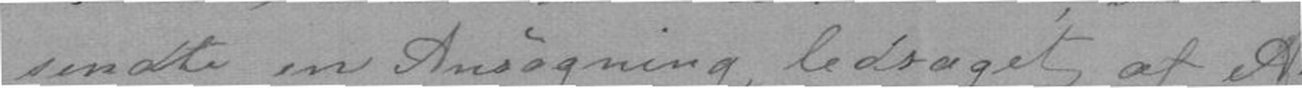jeg sendte en Ansøgning, ledsaget af Attes-
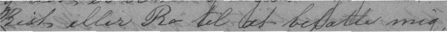Rist eller Ro til at befatte mig
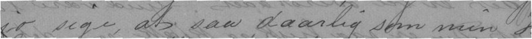jo sige, at saa daarlig som min
